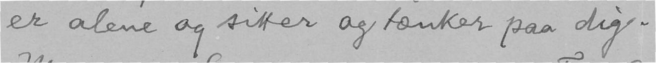er alene og sitter og tænker paa dig.
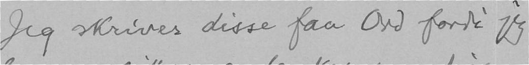Jeg skriver disse faa Ord fordi jeg
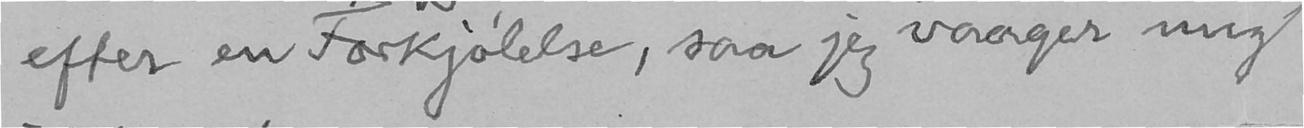efter en Forkjølelse, saa jeg vaager mig
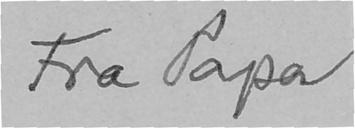Fra Papa
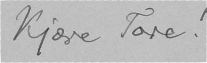Kjære Tore!
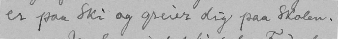er paa Ski og greier dig paa Skolen.
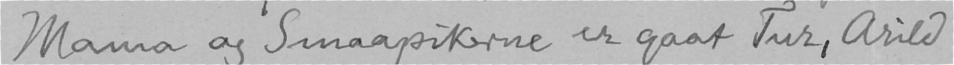Mama og Smaapikerne er gaat Tur, Arild
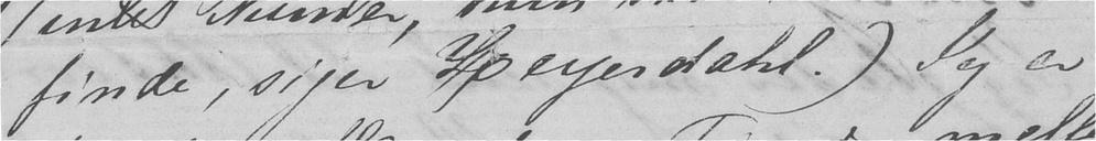finde, siger Heyerdahl.) Jeg er
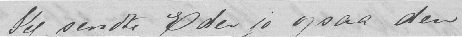Jeg sendte Eder jo ogsaa den
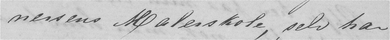nersens Malerskole, selv har
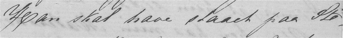Han skal have staaet paa Ste-
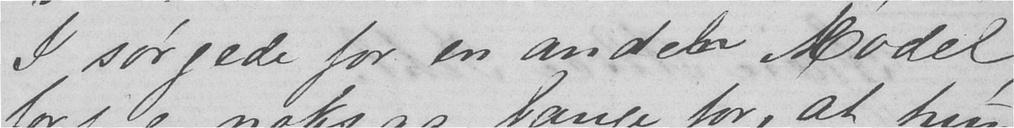I sørgede for en anden Model,
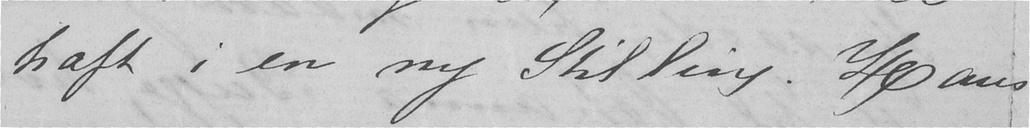haft i en ny Stilling. Hans
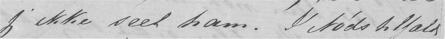jeg ikke seet ham. I Nødstilfælde
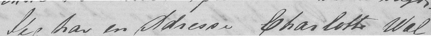Jeg har en Adresse Charlotte Wal-
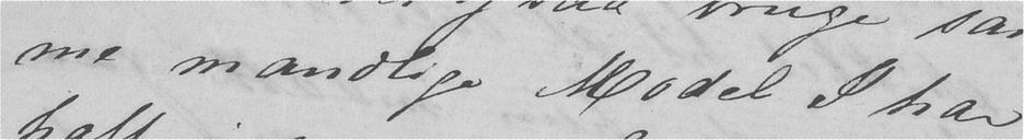me mandlige Model I har
